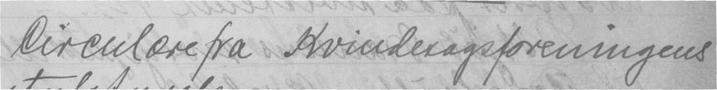Circulære fra Kvindesagsforeningens
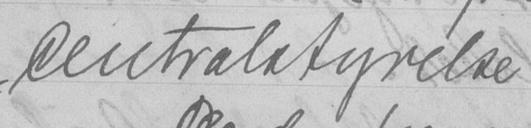centralstyrelse.
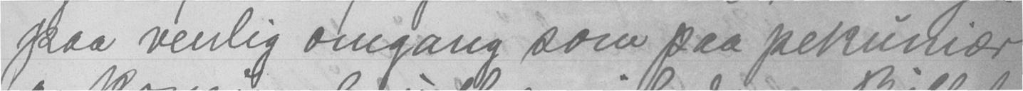paa venlig omgang som paa pekuniær
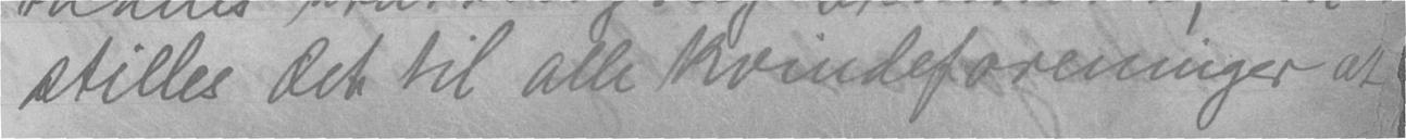stilles det til alle kvindeforeninger at
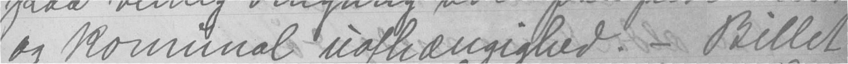og kommunal uafhængighed. - Billet
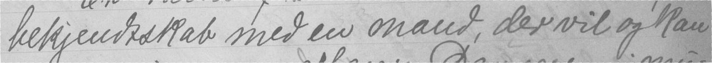bekjendtskab med en mand, der vil og kan
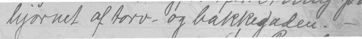hjørnet af torv- og bakkegaden. -
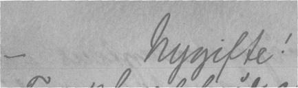- Nygifte!
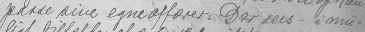passe sine egne affærer. Der seis - i mu-
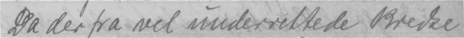Da der fra vel underrettede kredse
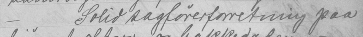- Solid sagførerforretning paa
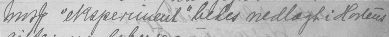med "eksperiment" bedes nedlagt i Hortens
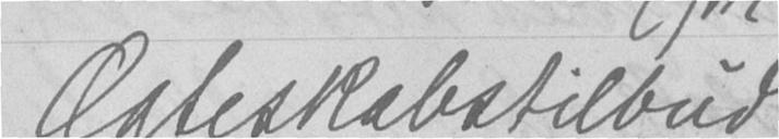Ægteskabstilbud
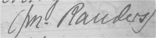(frk. Randers)
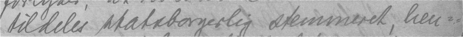tildeles statsborgerlig stemmeret, hen-
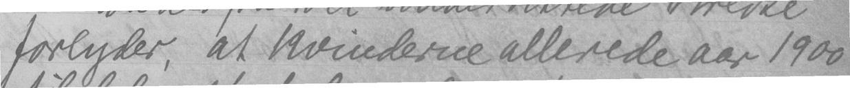forlyder, at kvinderne allerede aar 1900
